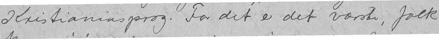Kristianiasprog. For det er det værste, folk
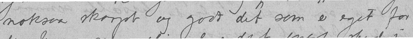noksaa skarpt og godt det som er eget for
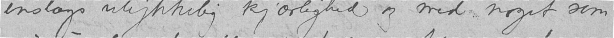enslags ulykkelig kjærlighed og med noget som
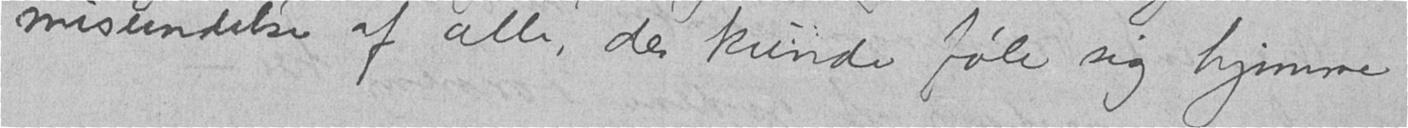misundelse af alle, der kunde føle sig hjemme
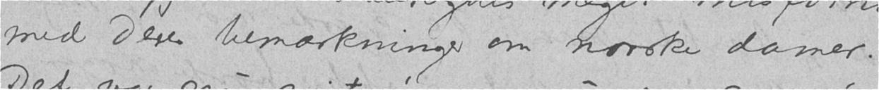med Dere bemærkninger om norske damer.
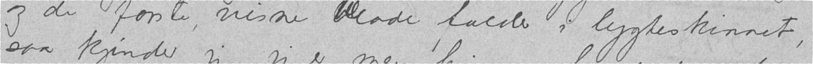og de første, visne blade falder i lygteskinnet,
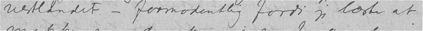vestlandet - formodentlig fordi jeg lærte at
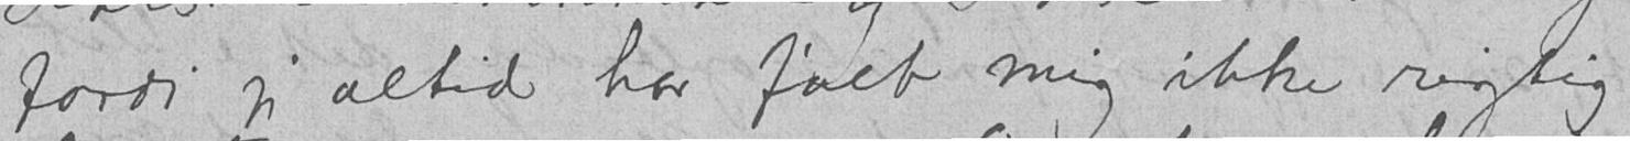fordi jeg altid har følt mig ikke rigtig
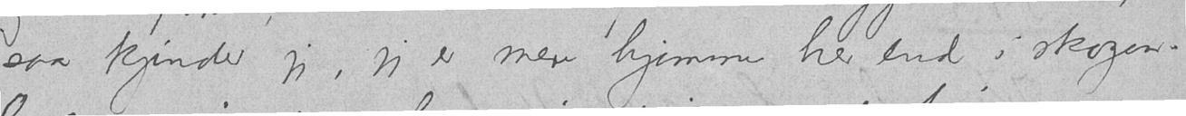saa kjender jeg, jeg er mere hjemme her end i skogen.
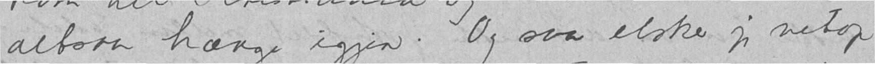altsaa hænge igjen. Og saa elsker jeg netop
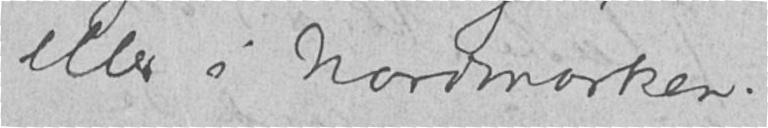eller i Nordmarken.
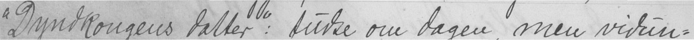"Dyndkongens datter": tudse om dagen, men vidun-
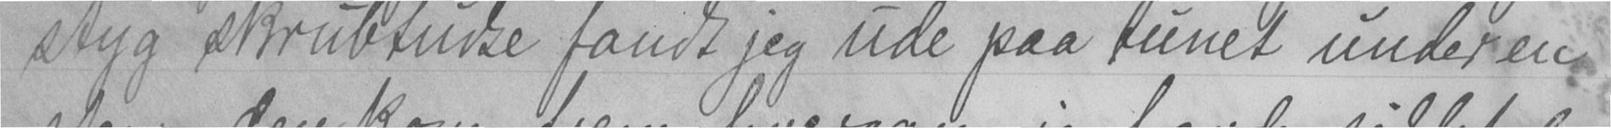styg skrubtudse fandt jeg ude paa tunet under en
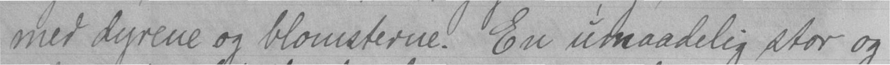med dyrene og blomsterne. En umaadelig stor og
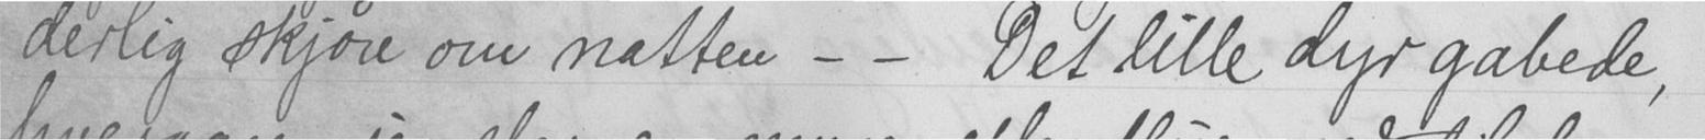derlig skjøn om natten - - Det lille dyr gabede,
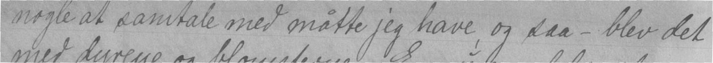nogle at samtale med måtte jeg have, og saa - blev det
